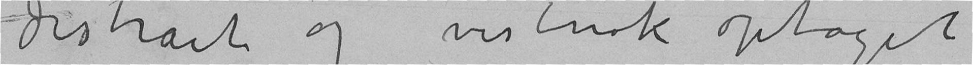distrait og vistnok optaget
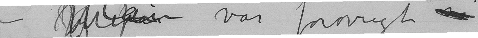– JegHan var forøvrigt så
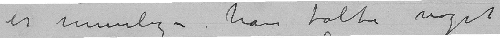er umulig – han talte noget
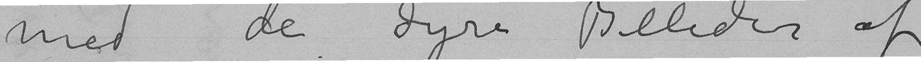med de dyre Billeder af
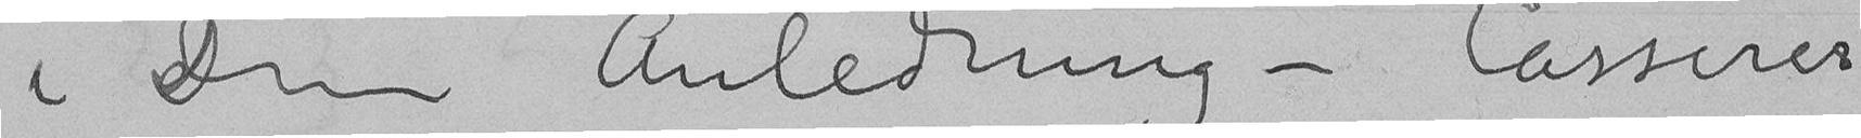i Din Anledning – Cassirer
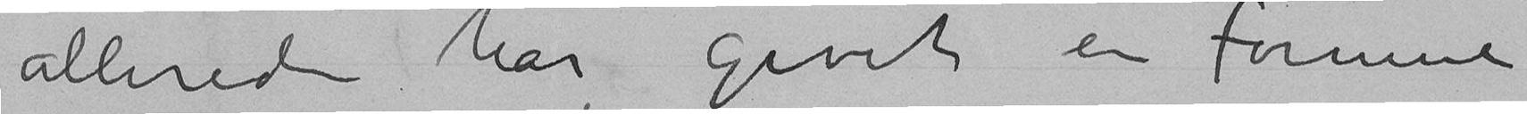allerede har givet en Formue
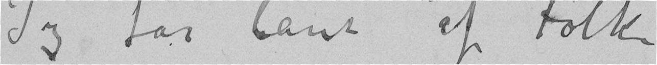Jeg får lånt af Folke
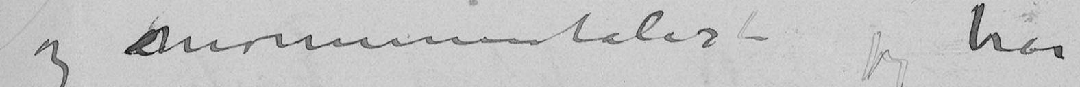og monumentaleste jeg har
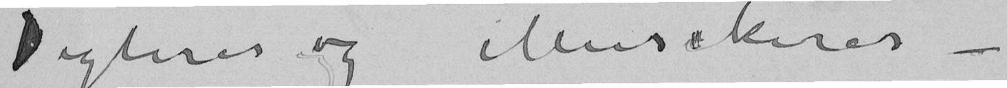Digteres og Musikeres –
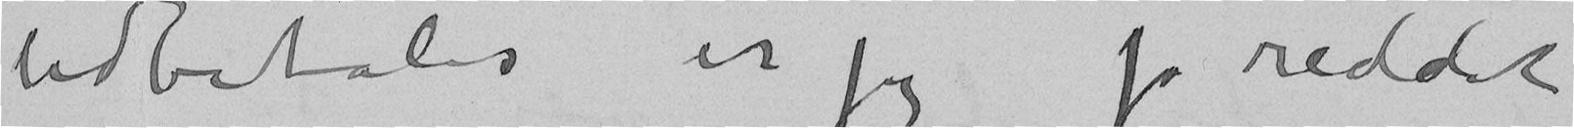udbetales er jeg jo reddet
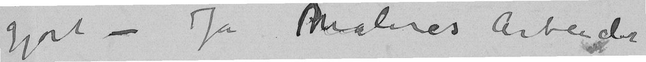gjort – Ja Maleres Arbeider
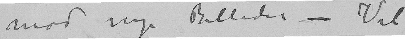mod nye Billeder – Vil
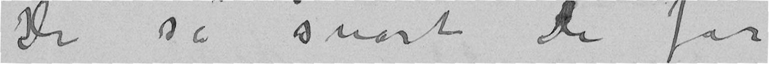De så snart De får
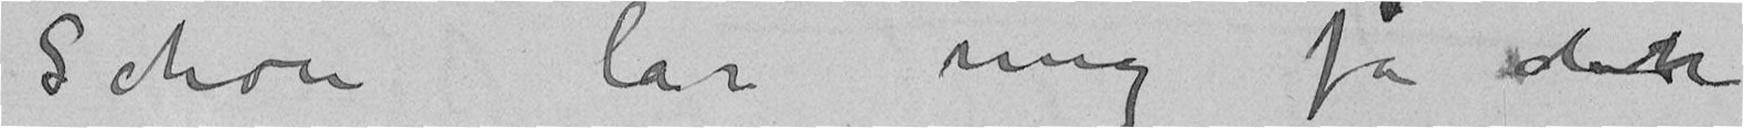Schou lar mig få den
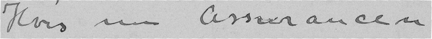Hvis nu Assurancen
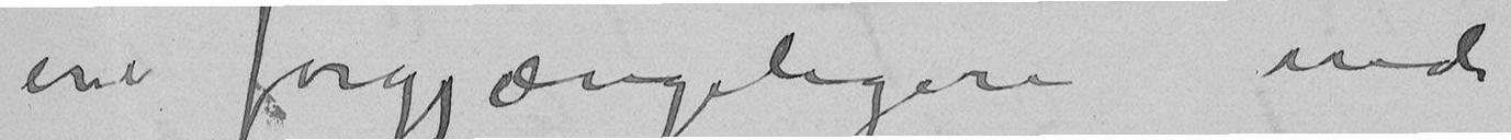ere forgjængeligere end
